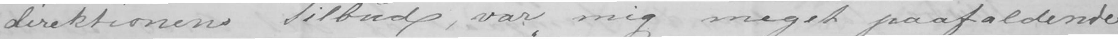direktionens Tilbud, var mig meget paafaldende,
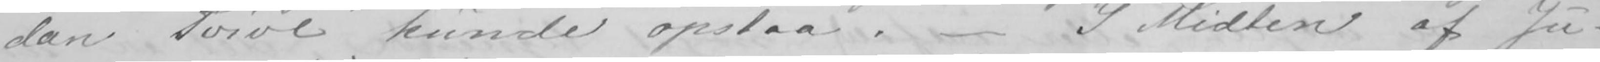dan Tvivl kunde opstaa.- I Midten af Ju-
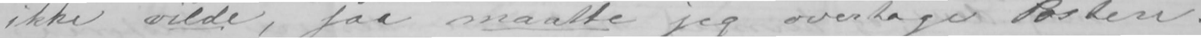ikke vilde, saa maatte jeg overtage Posten.
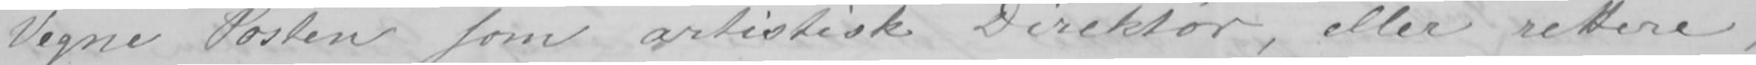Vegne Posten som artistisk Direktør, eller rettere,
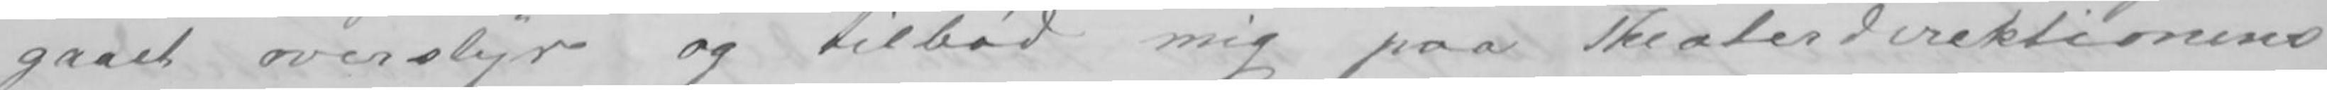gaaet overstyr og tilbød mig paa Theaterdirektionens
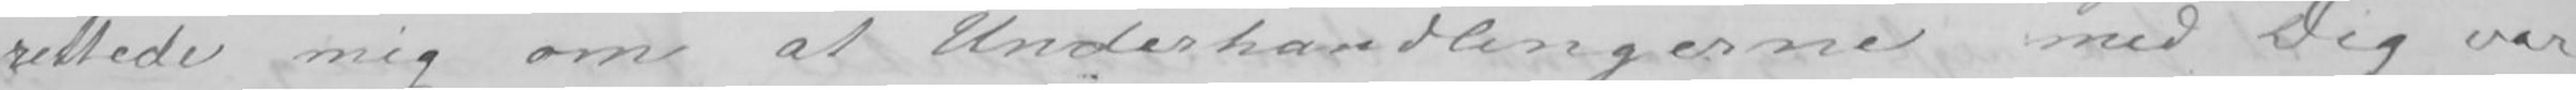rettede mig om at Underhandlingerne med Dig var
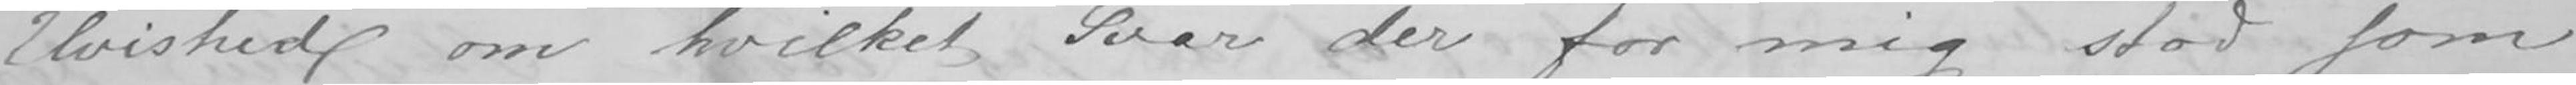Uvished om hvilket Svar der for mig stod som
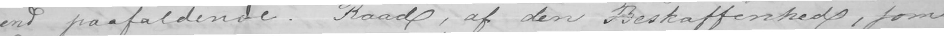end paafaldende. Raad, af den Beskaffenhed, som
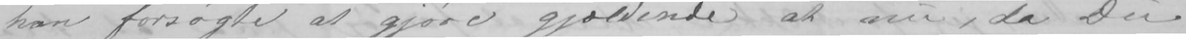han forsøgte at gjøre gjældende at nu, da Du
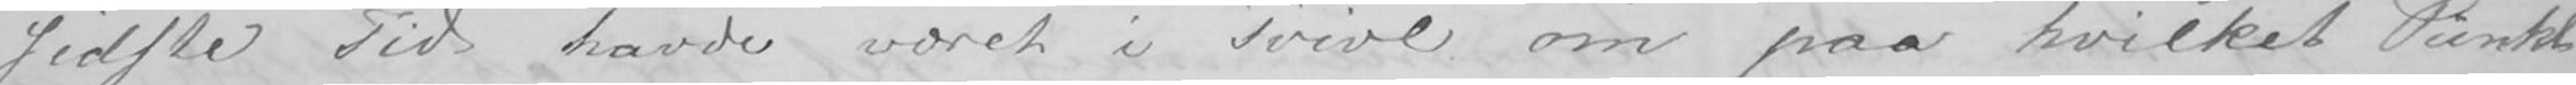sidste Tid havde været i Tvivl om paa hvilket Punkt
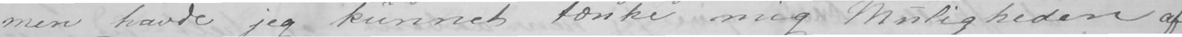men havde jeg kunnet tænke mig Muligheden af
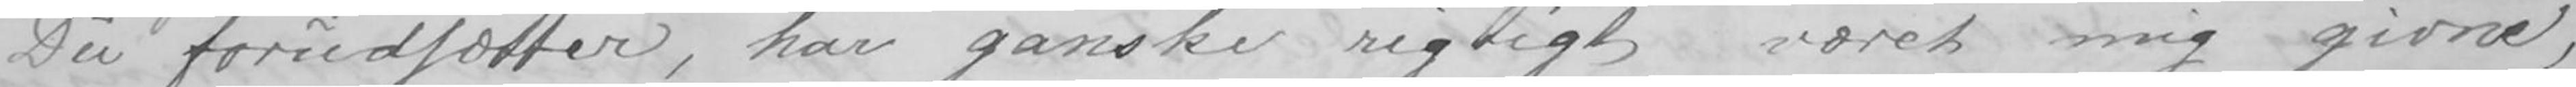Du forudsætter, har ganske rigtigt været mig givne,
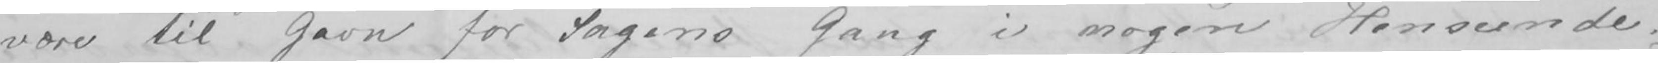være til Gavn for Sagens Gang i nogen Henseende;
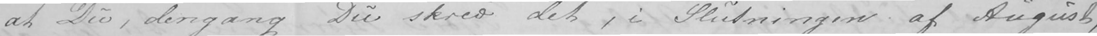at Du, dengang Du skrev det, i Slutningen af August,
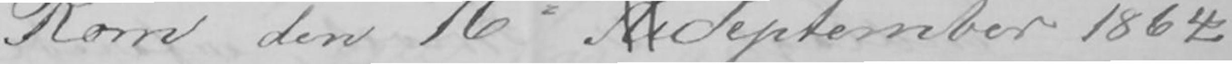Rom den 16de AuSeptember 1864.
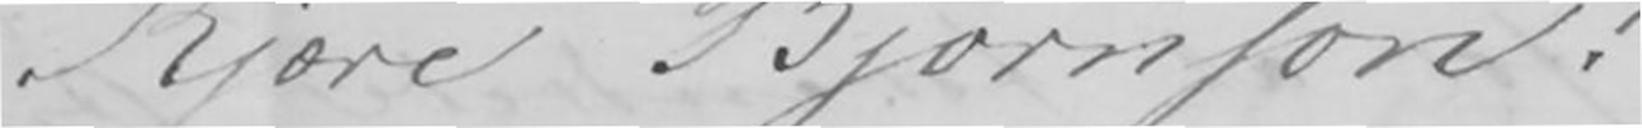Kjære Bjørnson!
